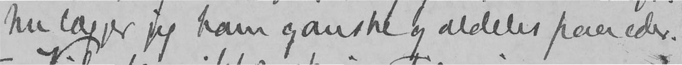Nu lægger jeg ham ganske og aldeles paa eder.
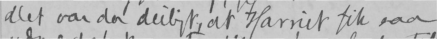Det var da deiligt at Harriet fik saa
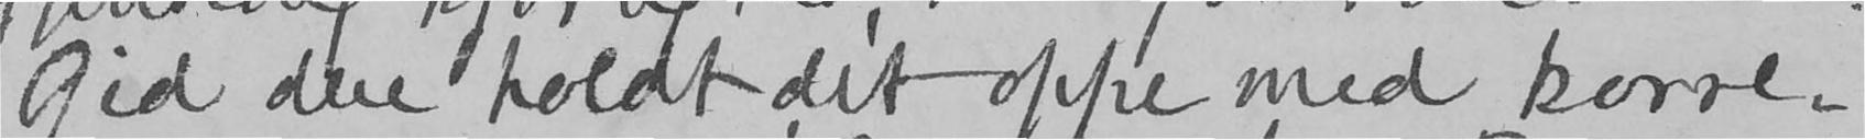Gid dere holdt det oppe med korre-
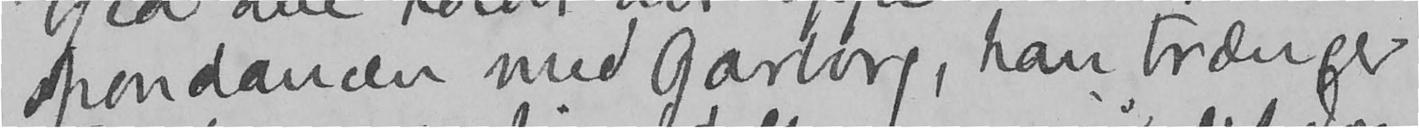spondancen med Garborg, han trænger
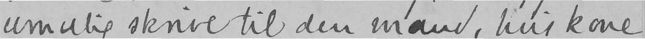umulig skrive til den mand, hvis kone
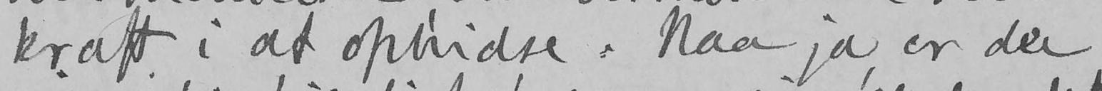kraft i at ophidse. Naa ja, er der
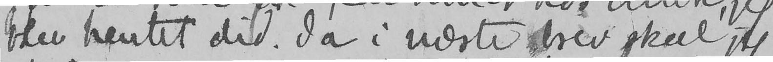blev hentet did. Ja i næste brev skal jeg
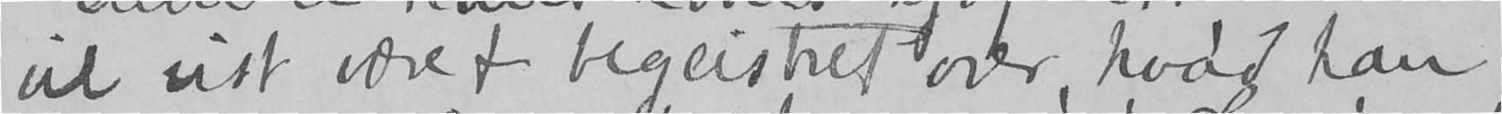vil vist været begeistret over, hvad han
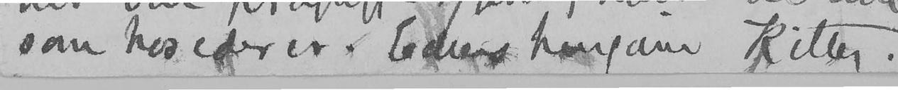som hos eder er. Eders hengivne Kitty.
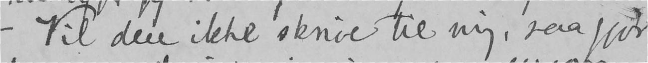- Vil dere ikke skrive til mig, saa gjør
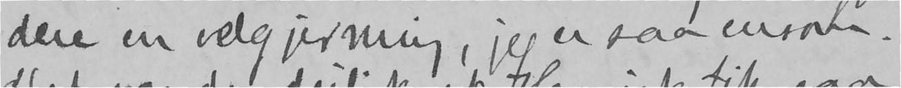dere en velgjerning, jeg er saa ensom.
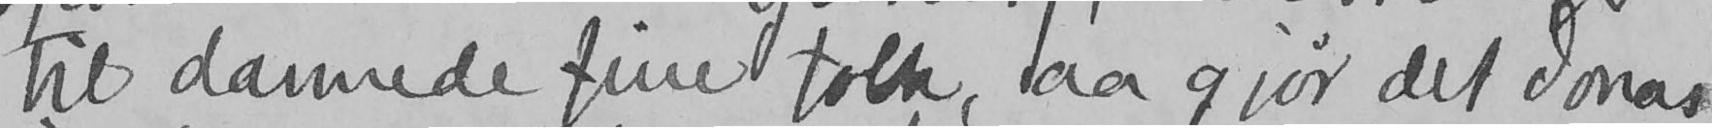til dannede fine folk, aa gjør det Jonas
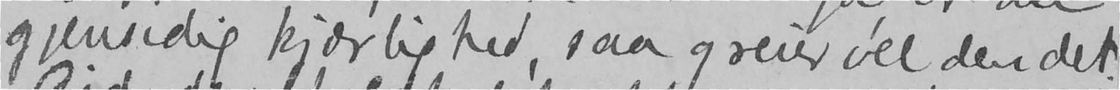gjensidig kjærlighed, saa greier vel den det.
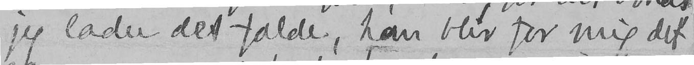jeg lader det falde, han blir for mig det
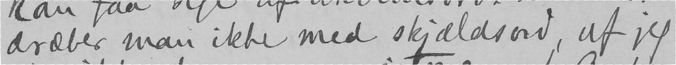dræber man ikke med skjældsord, uf jeg
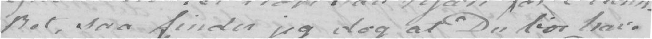ket, saa finder jeg dog at Du bør have
